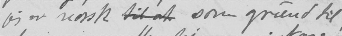jeg er norsk til at som grund til
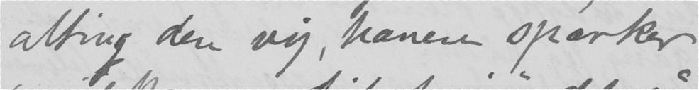alting den vej, hanen sparker
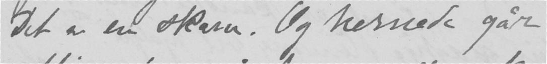Det er en Skam. Og hernede går
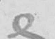e
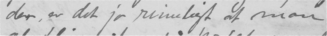der, er det jo rimeligt at man
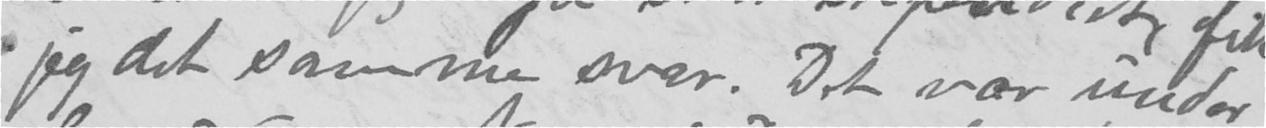jeg det samme svar. Det var under
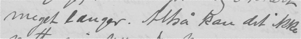meget længer. Altså kan det ikke
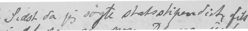Sidst da jeg søgte statsstipendiet, fik
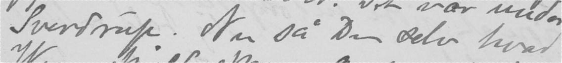Sverdrup. Nu så De selv hvad
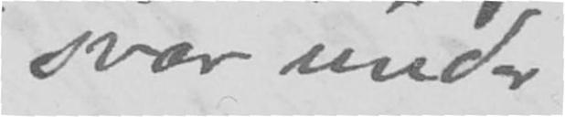svar under
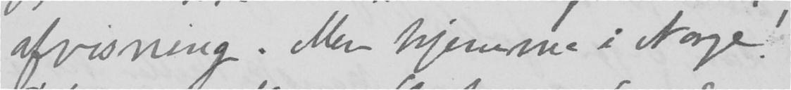afvisning. Men hjemme i Norge!
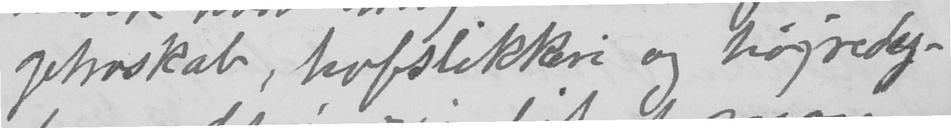getroskab, hofslikkeri og højredy-
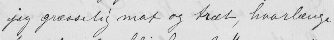jeg græsselig mat og træt, hvorlænge
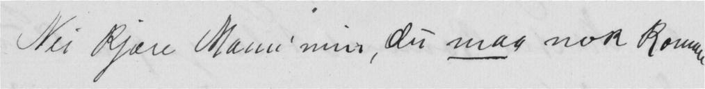Nei Kjære Mann min, du maa nok Komme
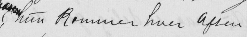hun Kommer hver Aften
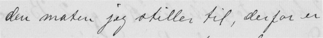den maten jeg stiller til, derfor er
